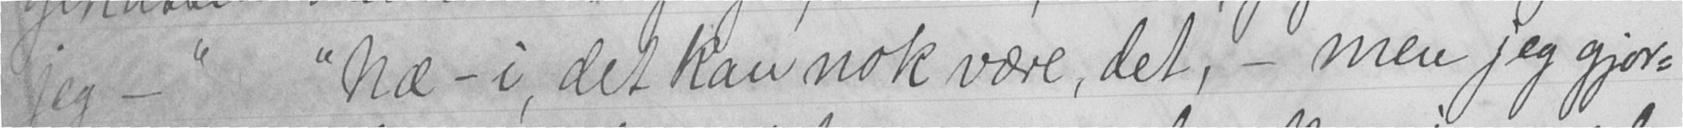jeg - " "Næ-i, det kan nok være, det, - men jeg gjor-
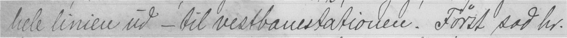hele linien ud - til vestbanestationen. Først sad hr.
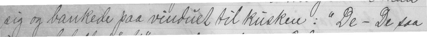sig og bankede paa vinduet til kusken: "De - De saa
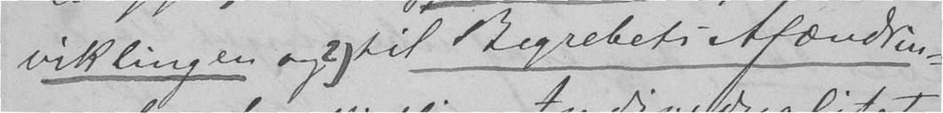viklingen og til Begrebets Afændrin-
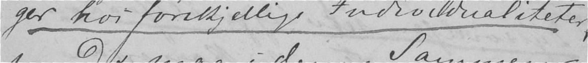ger hos forskjellige Individualiteter;
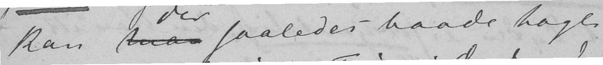kan saaledes baade tages
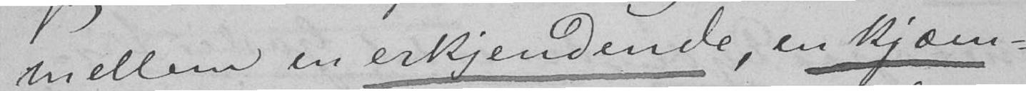mellem en erkjendende, en kjæm-
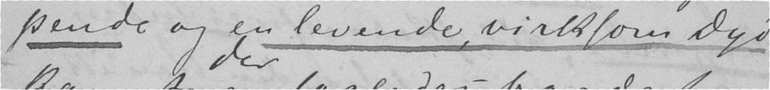pende og en levende, virksom Dyd,
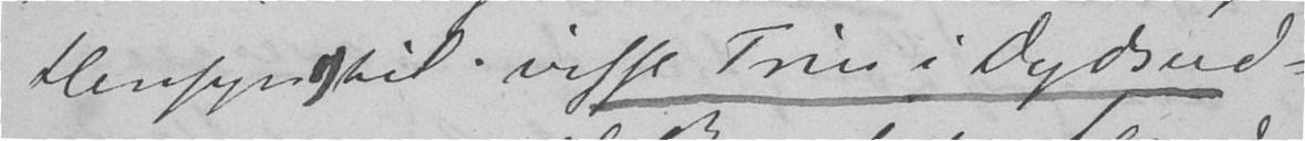Hensyn 1) til visse Trin i Dydsud-
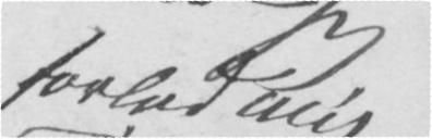forlod mig
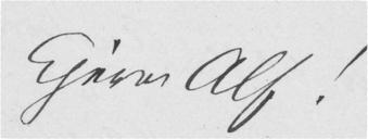Kjere Alf!
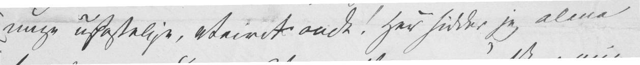unge utastelige, Veiret ondt! Her sidder jeg alene
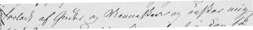forladt af Guder og Mennesker og ønsker mig
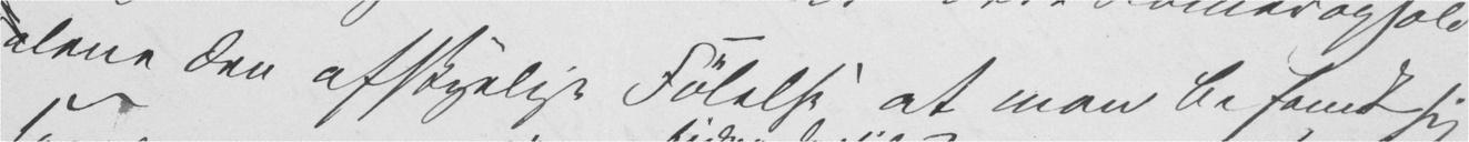alene den afskyelige Følelse at man befandt sig
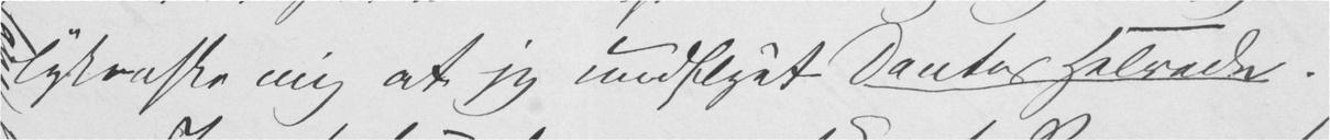lykønske mig at jeg undflyet Dantes Helvede.
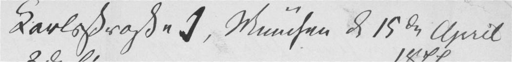Karlsstrasse 1, München d 15de April
